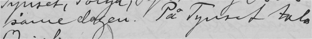same dagen. På Tynset tala
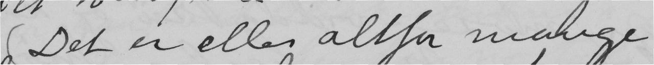Det er elles altfor mange
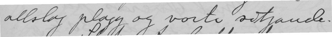allslag plagg og vorte sitjande.
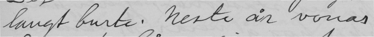langt burte. Neste år vonar
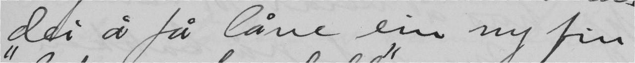dei å få låne ein ny fin
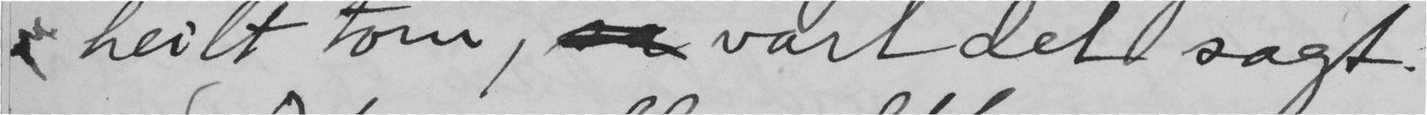heilt tom,  vart det sagt.
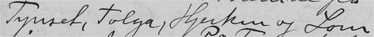Tynset, Tolga, Hjerkin og Lom
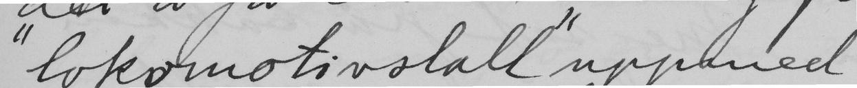"lokomotivstall" uppmed
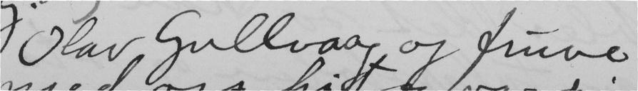Olav Gullvaag og fruve
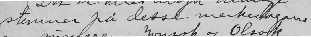stemner på desse merkedagane
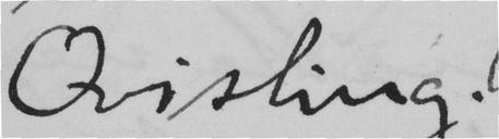Qvisling.
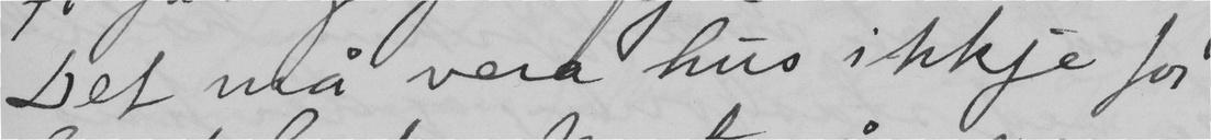Det må vera hus ikkje for
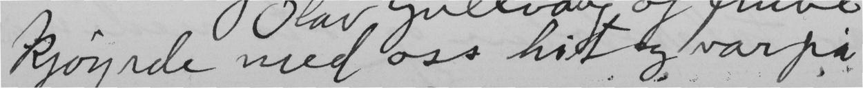kjöyrde med oss hit og varpå
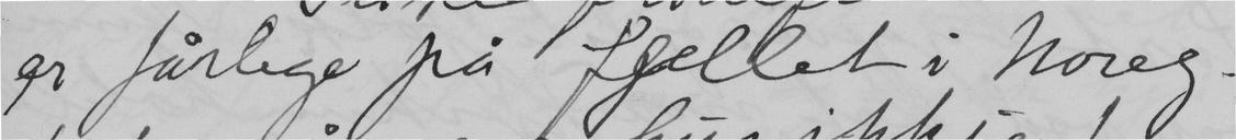er fårlege på fjellet i Noreg.
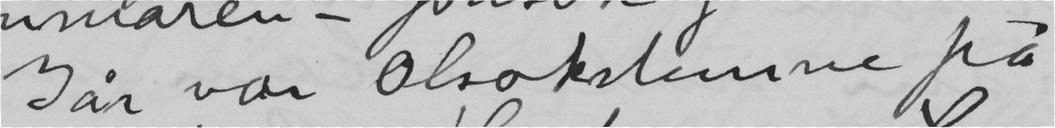I år var olsokstemne på
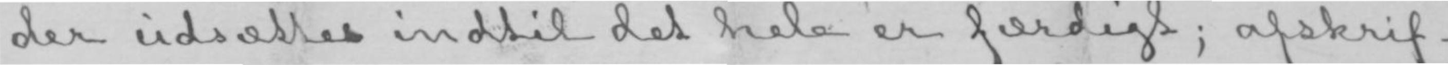der udsættes indtil det hele er færdigt; afskrif-
